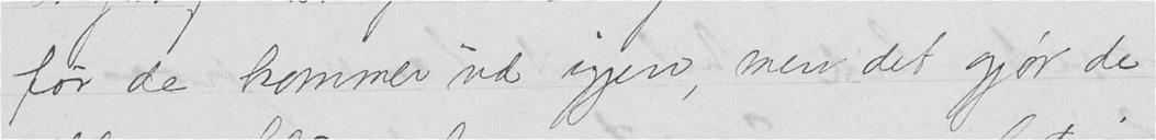før de kommer ud igjen, men det gjør de
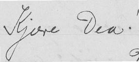Kjære Dea!
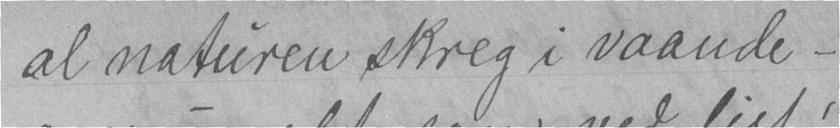al naturen skreg i vaande -
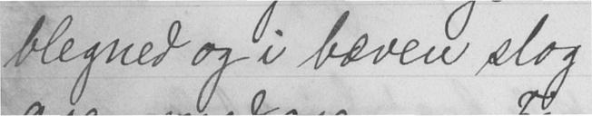blegned og i bæven slog
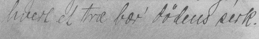hvert et træ bær' dødens serk.
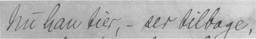nu han tier, - ser tilbage,
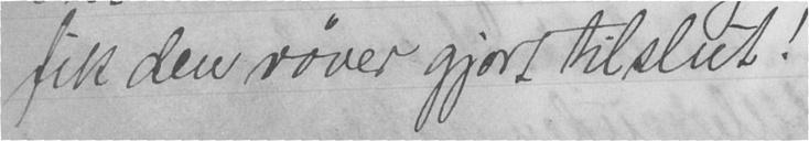fik den røver gjort tilslut!
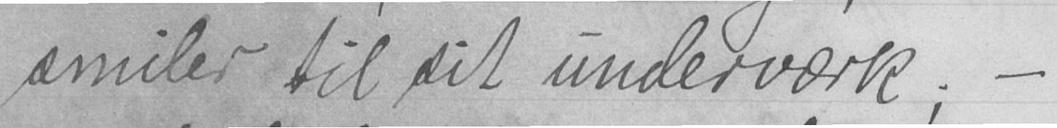smiler til sit underværk; -
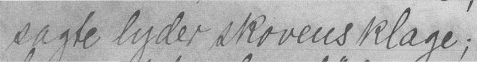sagte lyder skovens klage;
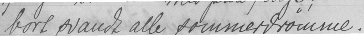vort svandt alle sommerdrømme;
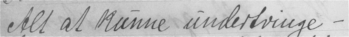Alt at kunne undertvinge -
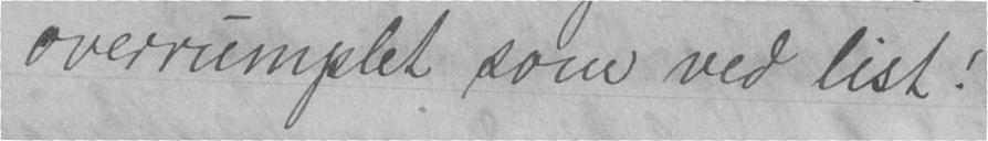overrumplet som ved list!
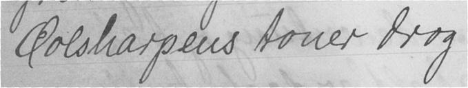Colsharpens toner drog
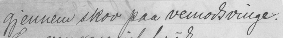gjennem skov paa vemodsvinge.
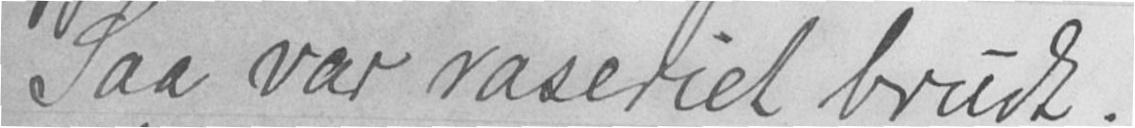Saa var raseriet brudt.
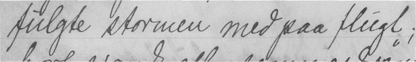fulgte stormen med paa flugt;
